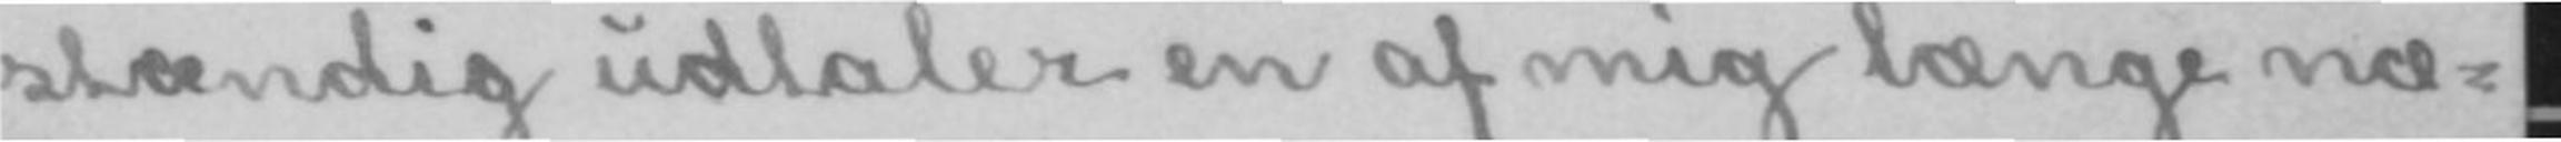stændig udtaler en af mig længe næ-
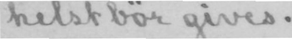helst bør gives.
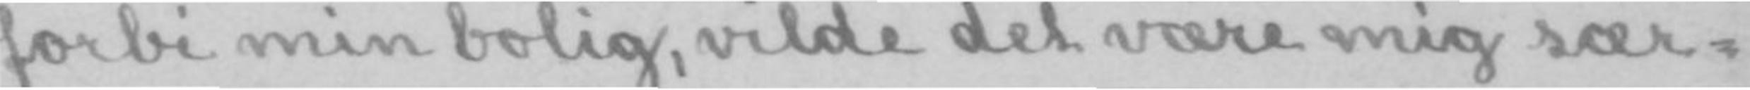forbi min bolig, vilde det være mig sær-
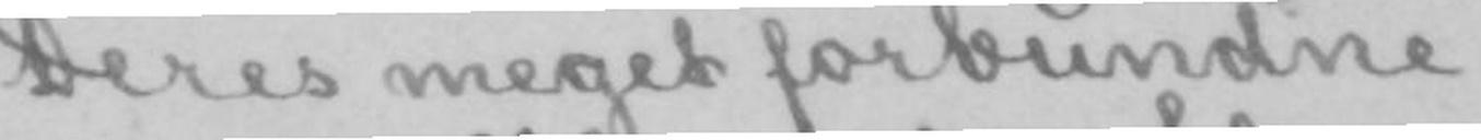Deres meget forbundne
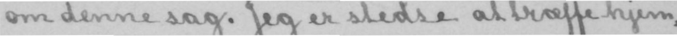om denne sag. Jeg er stedse at træffe hjem-
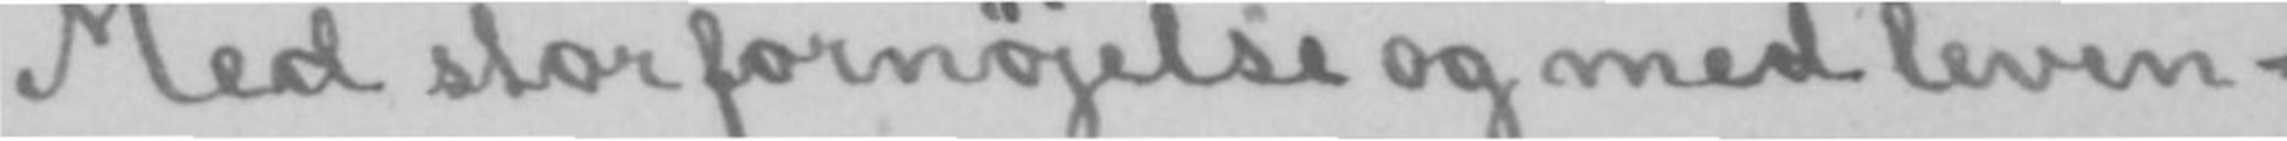Med stor fornøjelse og med leven-
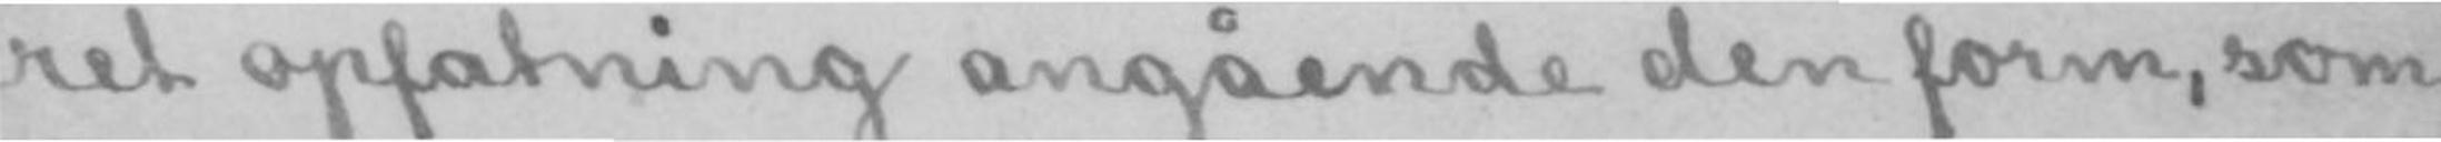ret opfatning angående den form, som
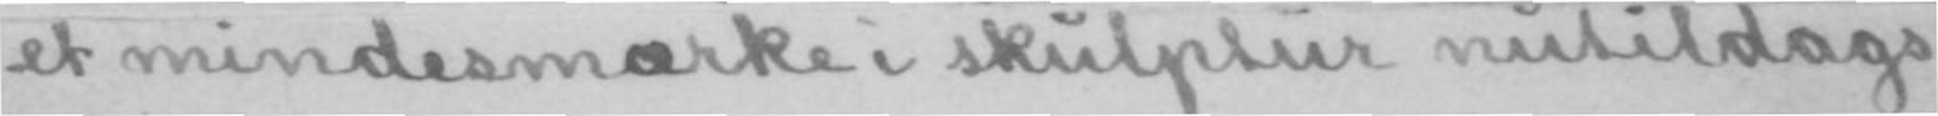et mindesmærke i skulptur nutildags
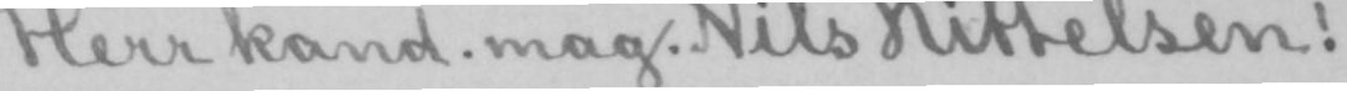Herr kand. mag. Nils Kittelsen!
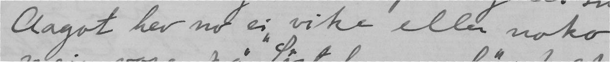Aagot hev nå ei vike eller noko
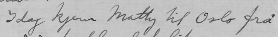Idag kjem Matty til Oslo frå
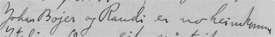Johan Bojer og Randi er no heimkomne
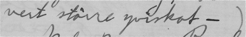vert större yviskot –
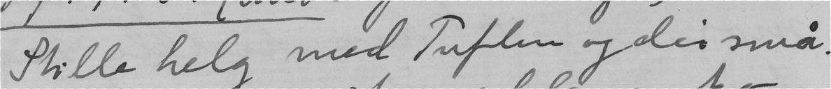Stille helg med Tuften og dei små.
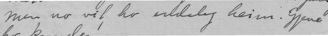Men no vil ho eideleg heim. Gjeve
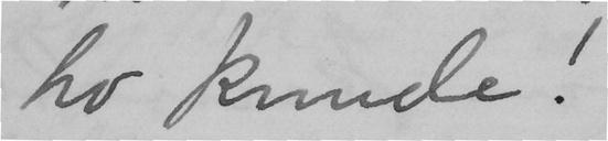ho kunde!
